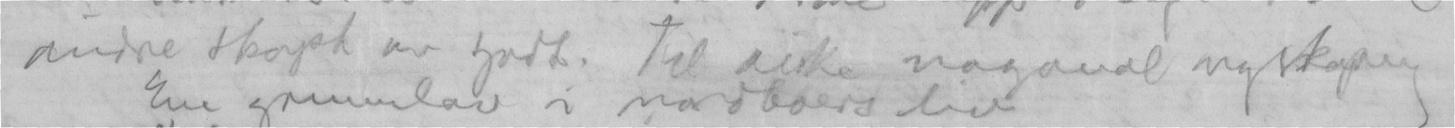andre skapt av godt. Til virke nogaval nyskaping
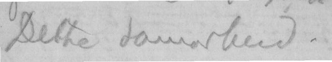Dette samarbeid.
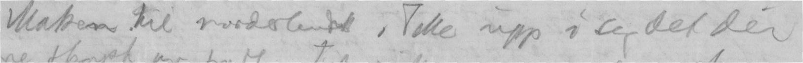Maken til norderlendi, teke upp i seg det dei
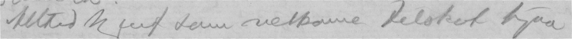Alltid kjent som velkomne tilskot hjaa
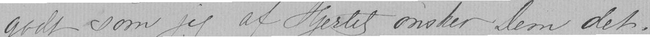godt som jeg af Hjertet ønsker Dem det.
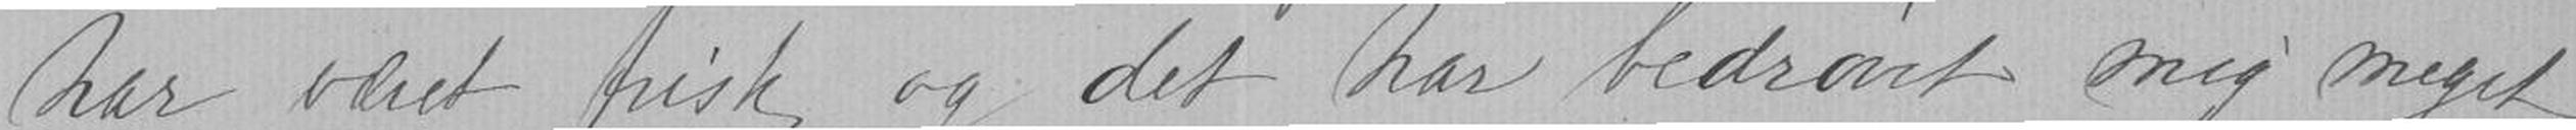har været frisk og det har bedrøvet mig meget
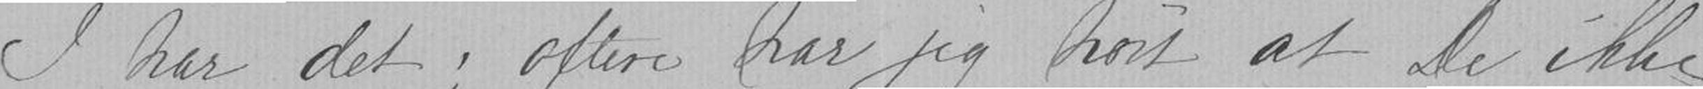I har det; oftere har jeg hørt at De ikke
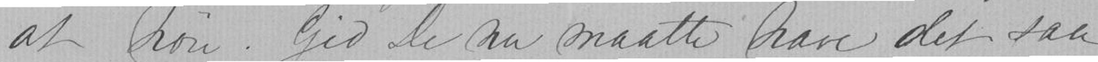at høre. Gid De nu maatte have det saa
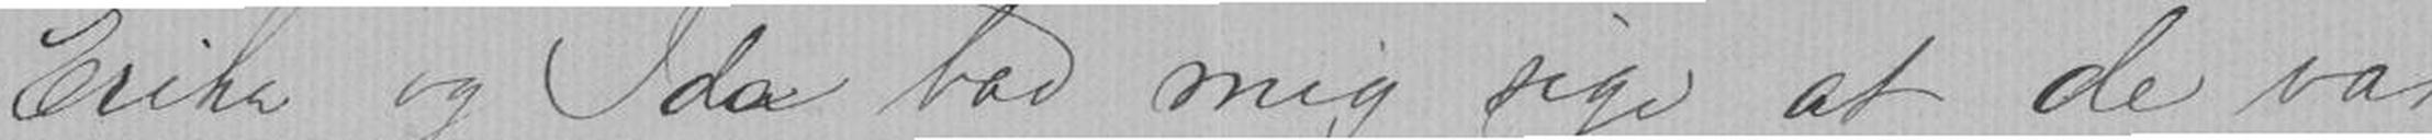Erika og Ida bad mig sige at de var
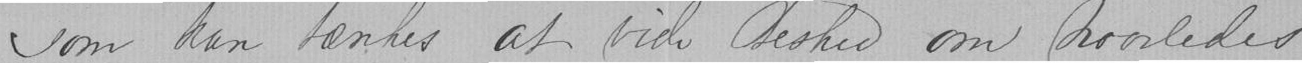som kan tænkes at vide Besked om hvorledes
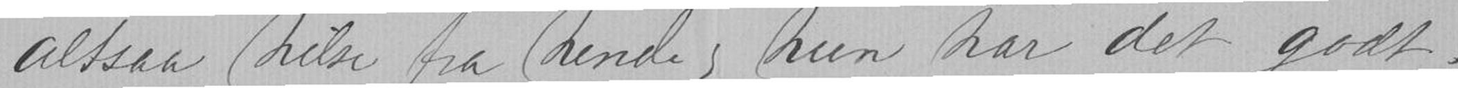altsaa hilse fra hende, hun har det godt.
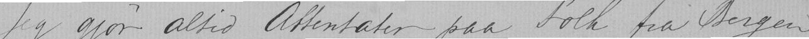Jeg gjør altid Attentater paa Folk fra Bergen
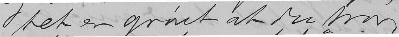tet er grønt at du tror,
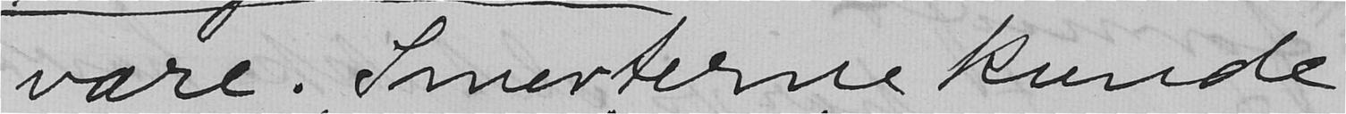være. Smerterne kunde
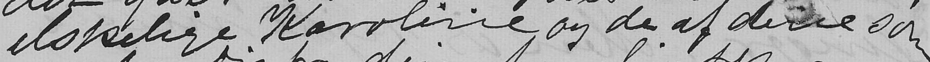elskelige Karoline og de af dine som
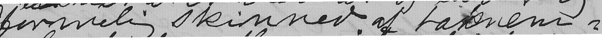formelig skinned af taknem-
